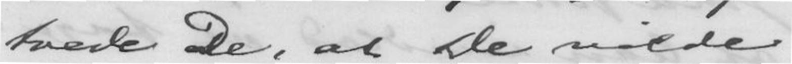rede De, at De vilde
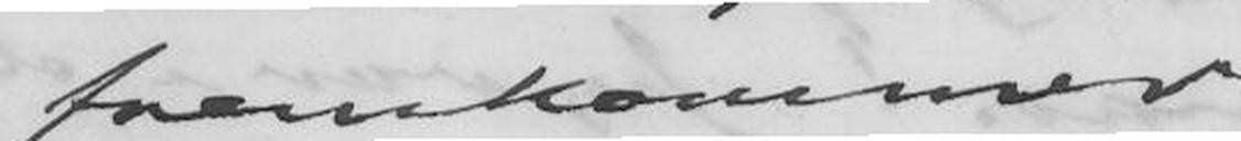fremkommer.
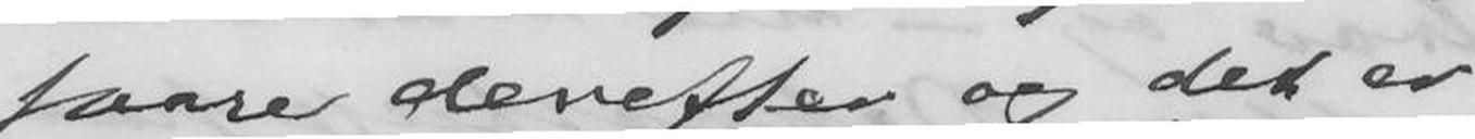saare derefter og det er
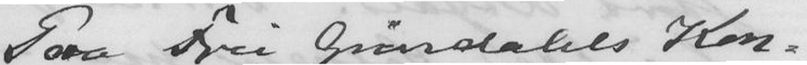Paa Fru Grøndahls Kon-
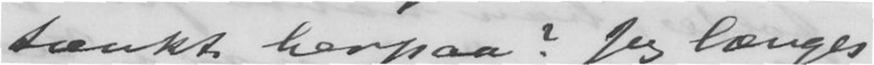tænkt herpaa? Jeg længes
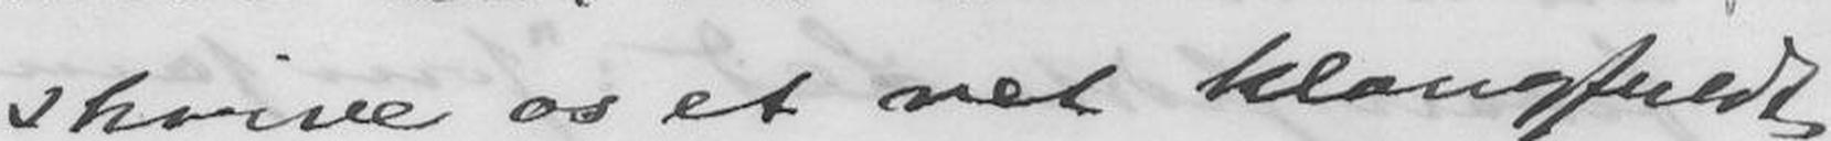skrive os et ret klangfuldt
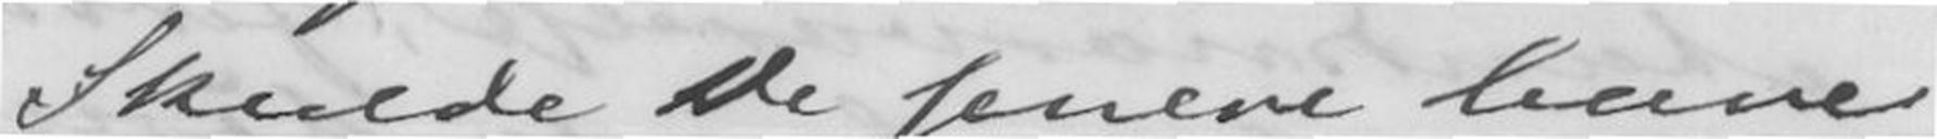Skulde De senere have
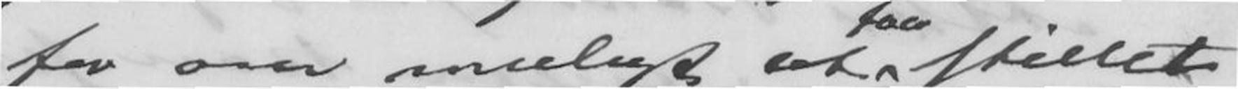for om muligt at stillet
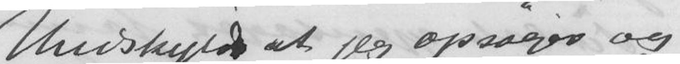Undskyld at jeg opsøger og
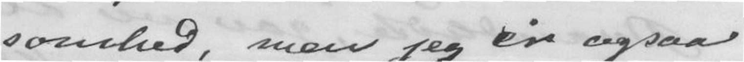somhed, men jeg er ogsaa
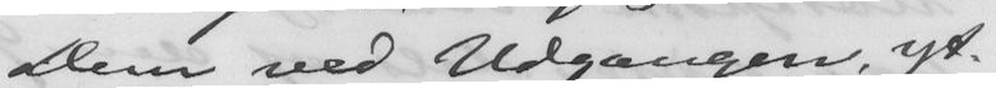Dem ved Udgangen, yt-
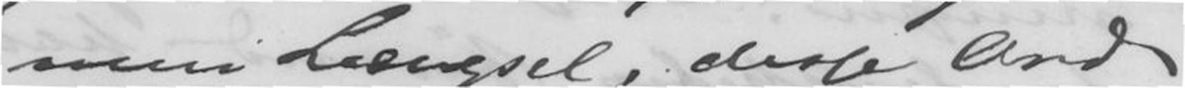min Længsel, disse Ord
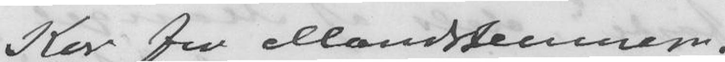Kor for Mandstemmer.
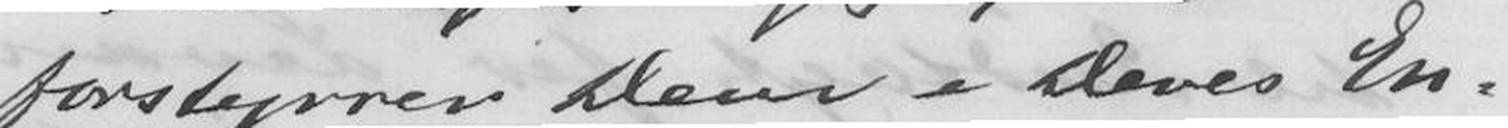forstyrrer Dem i Deres En-
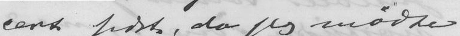cert sidst, da jeg mødte
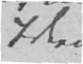Ideen
